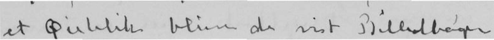et Øieblik bliver de vist Billedbøger
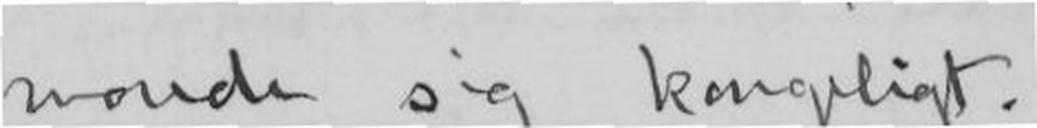vorde sig kongeligt.
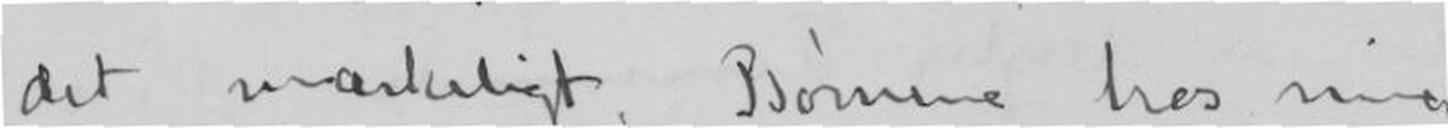det makeligt, Børnene hos mig
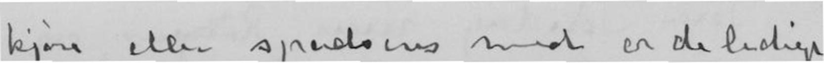kjøre, eller spadseres med, er de ledige
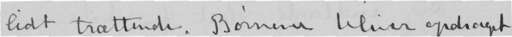lidt trættende. Børnene bliver opdaget
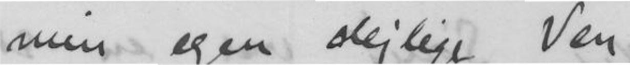min egen dejlige Ven,
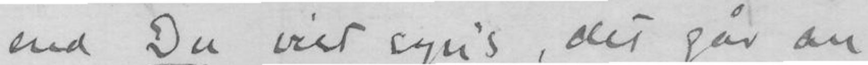end Du vist syn's, det går an.
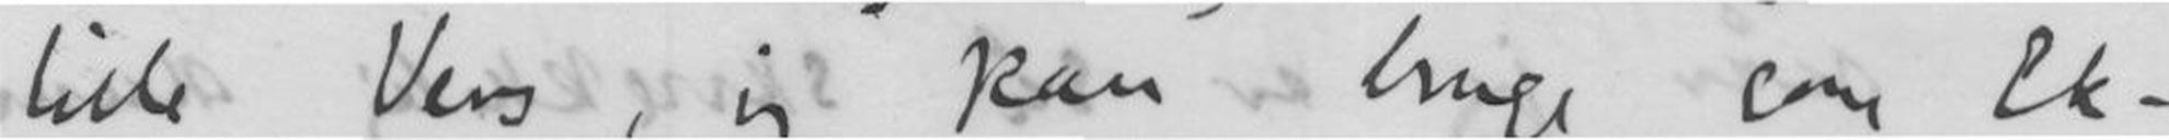lide Vers, jeg kan bruge som Ek-
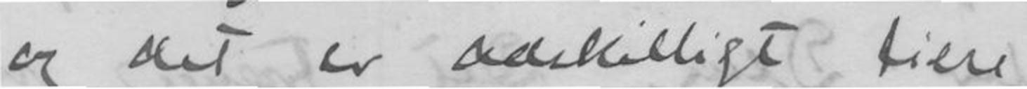og det er adskilligt tilse
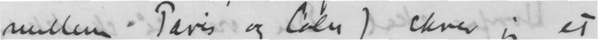mellem Paris og Cøln) skrev jeg et
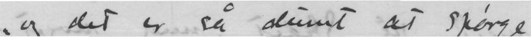og det er så dumt at spørge
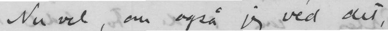Nu vel, om også jeg vèd det,
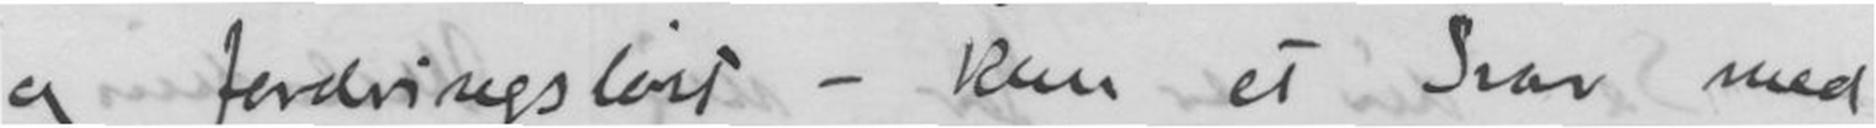og fordringsløst - kun et Svar med
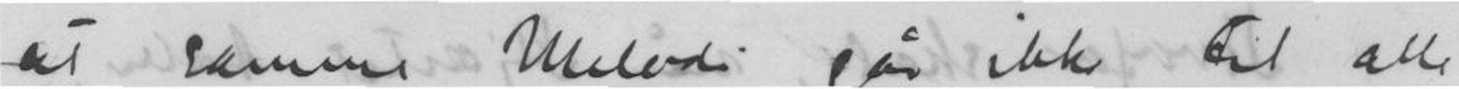at samme Melodi går ikke til alle
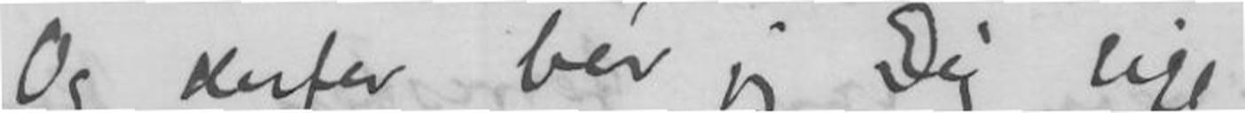Og derfor bèr jeg Dig sige
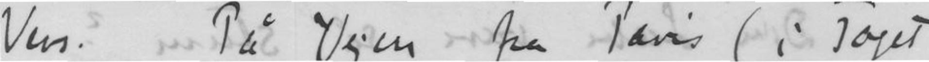Vers. På Vejen fra Paris (i Toget
